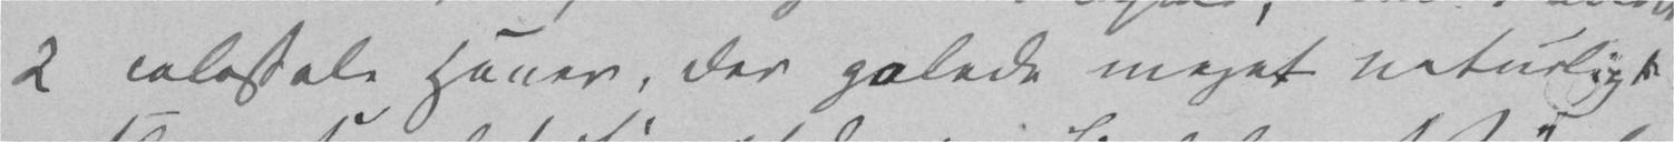2 colossale Haner, der galede meget naturligt
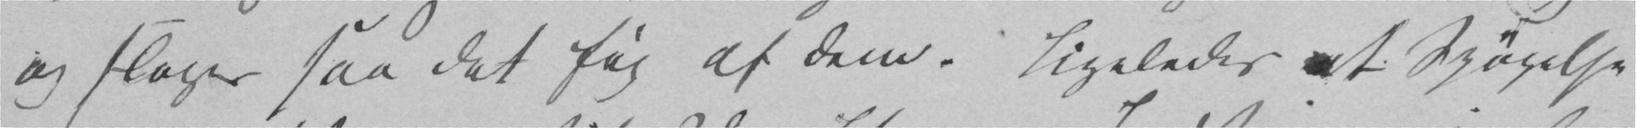og sloges saa det føg af dem. Ligeledes et Spøgelse
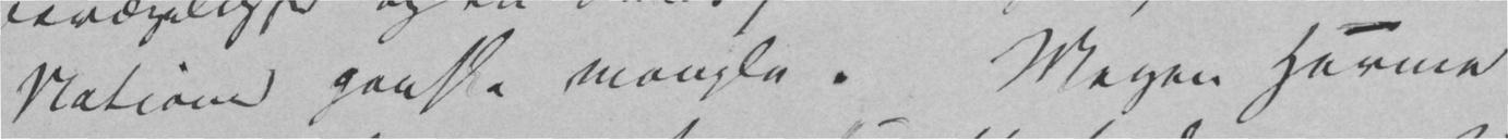Nationer ganske mangle. Megen Harme
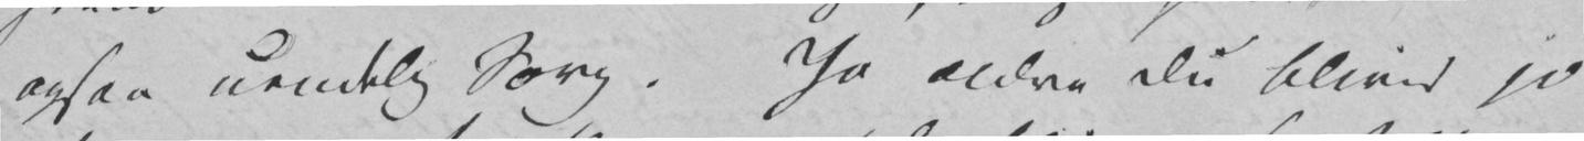ogsaa uendelig Sorg. Jo ældre Du bliver jo
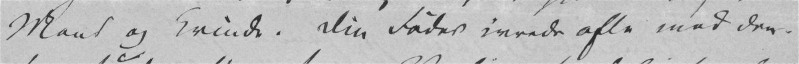Mand og Kvinde. Din Fader ivrede ofte mod den –
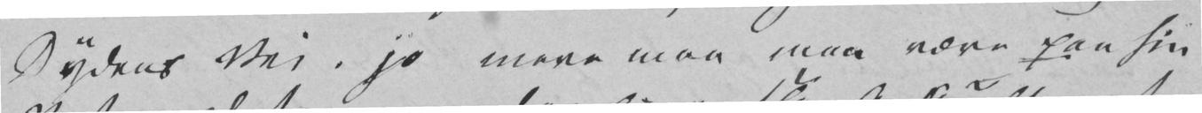Dydens Vei, jo mere maa man være paa sin
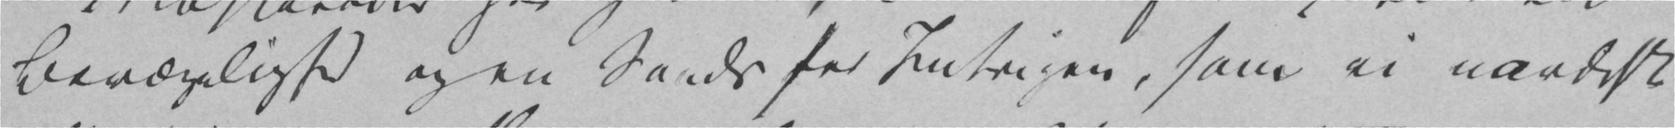Bevægelighed og en Sands for Intrigen, som vi nordiske
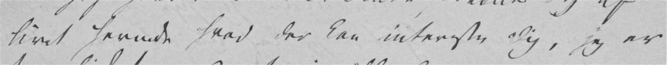Livet hernede hvad der kan interessere Dig, jeg er
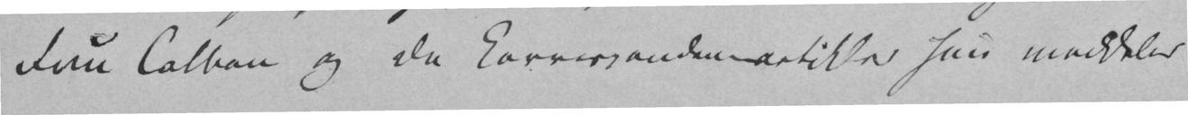Fru Colban og de Korrespondenceartikler hun meddeler
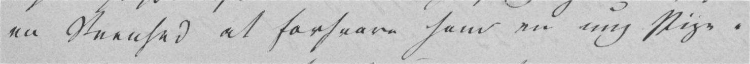en Reenhed at forsvare som en ung Pige.
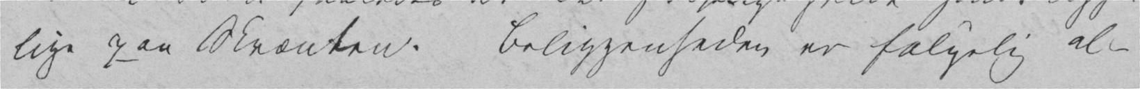lige paa Skrænten. Beliggenheden er følgelig al-
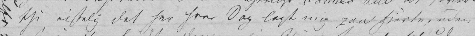thi visselig det har hver Dag lagt mig paa Hjerte, uden
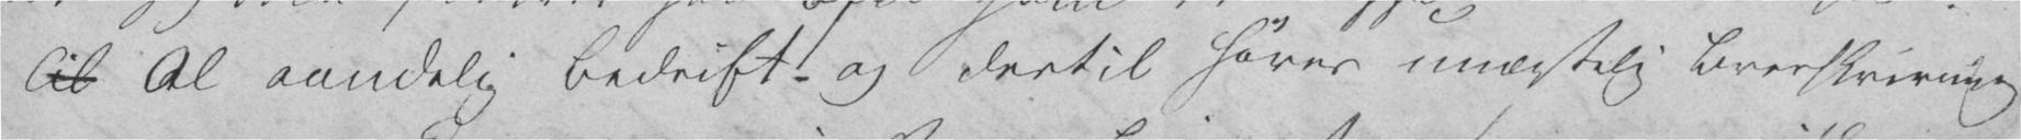Til Al aandelig Bedrift – og dertil hører unægtelig Brevskrivning,
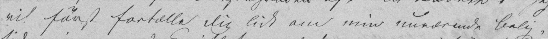vil først fortælle Dig lidt om min nuværende Bolig,
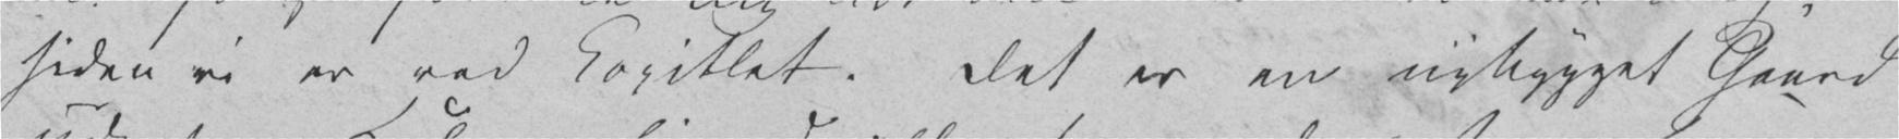siden vi er ved Kapitlet. Det er en nybygget Gaard
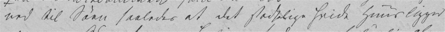saaledes at det stadselige hvide Huus ligger
